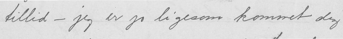tillid - jeg er jo ligesom kommet dig
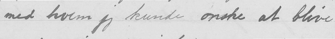med hvem jeg kunde ønske at blive
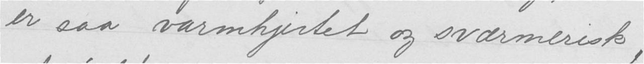er saa varmhjertet og sværmerisk,
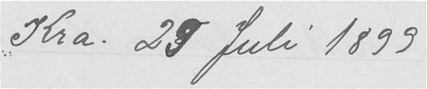Kra. 29. Juli 1899
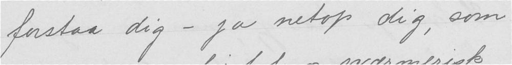forstaa dig - ja netop dig, som
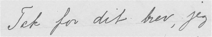Tak for dit brev, jeg
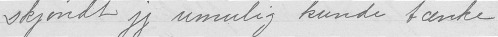skjøndt jeg umulig kunde tænke
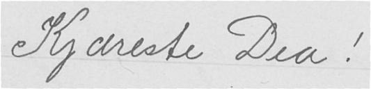Kjæreste Dea!
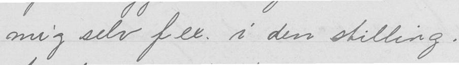mig selv f ex. i den stilling.
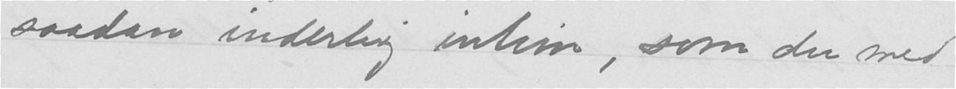saadan inderlig intim, som du med
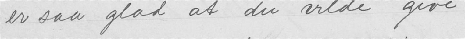er saa glad at du vilde give
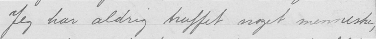Jeg har aldrig truffet noget menneske,
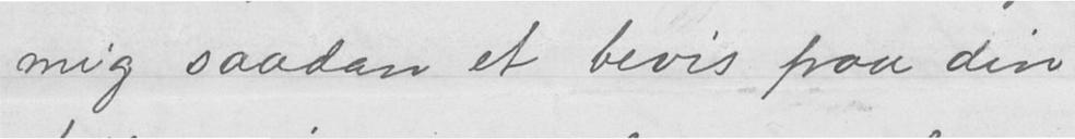mig saadan et bevis paa din
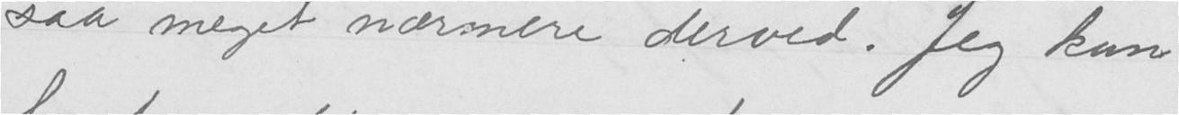saa meget nærmere derved. Jeg kan
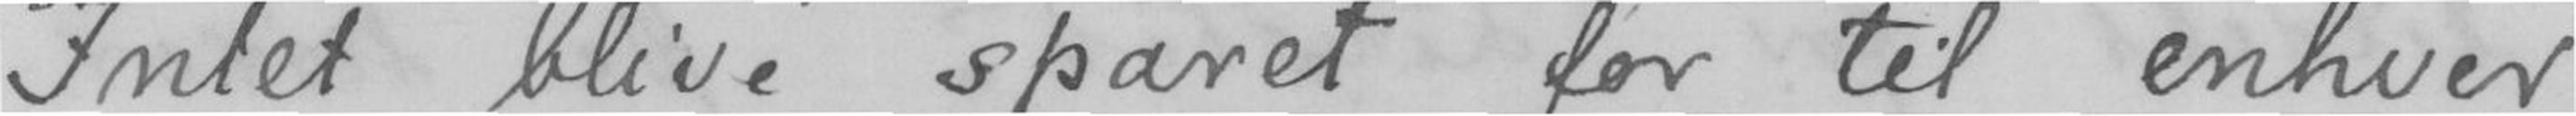Intet blive sparet for til enhver
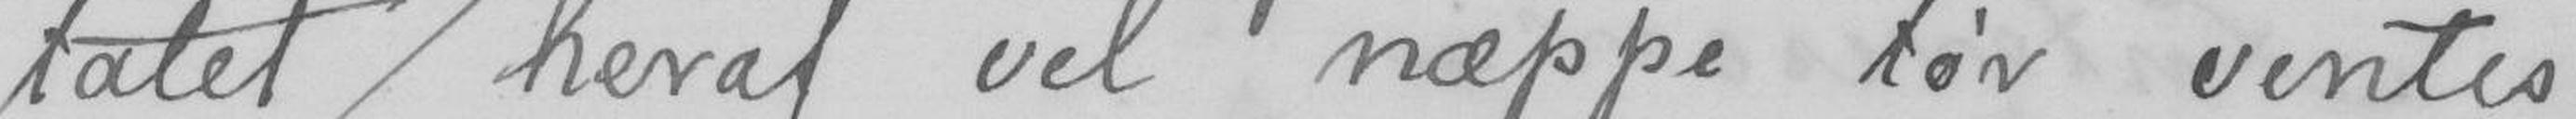tatet heraf vel næppe tør ventes
